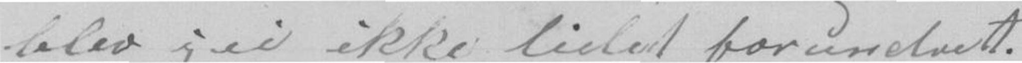blev jei ikke lidet forundret.
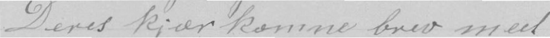Deres kjærkomne brev med
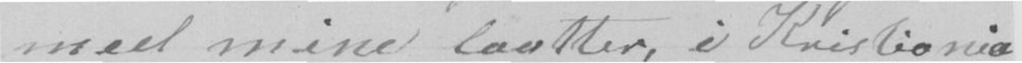med mine laatter, i Kristiania
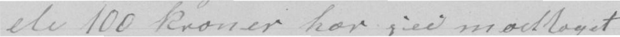de 100 kroner har jei modtaget
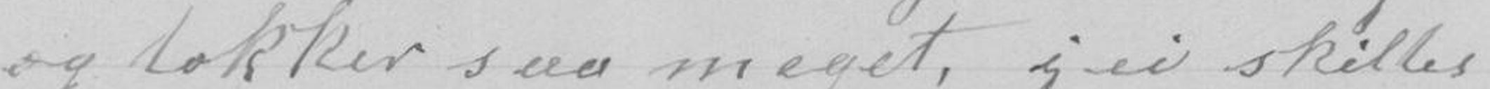og tokker saa meget, jei skiltes
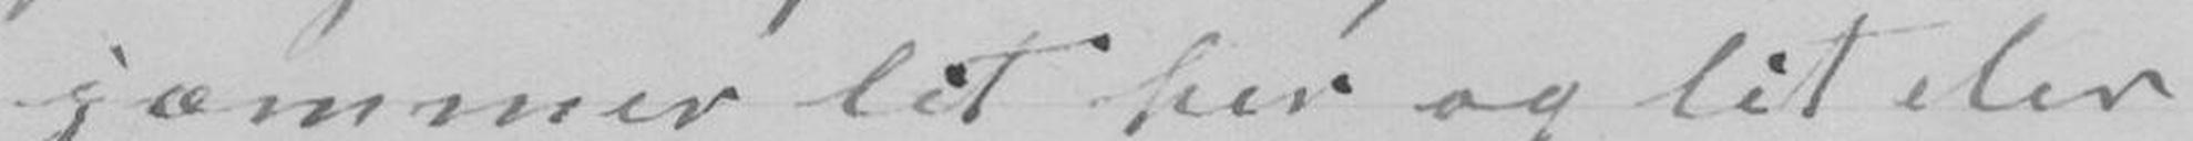jæmmer lit her og lit der
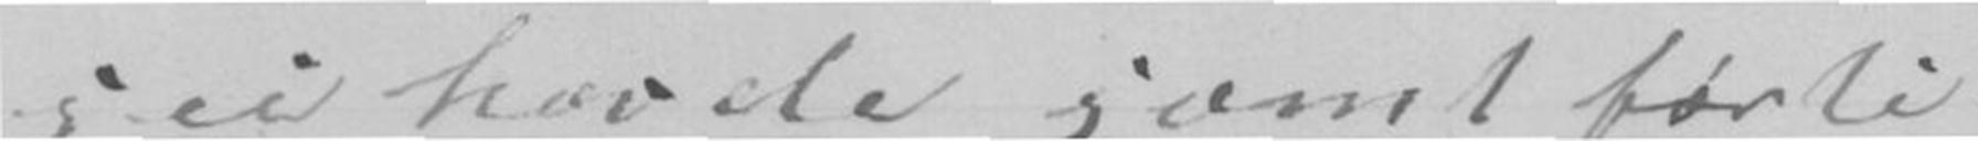jei havde jæmt førti
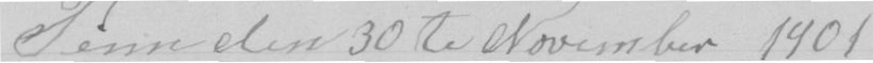Tinn den 30te November 1901
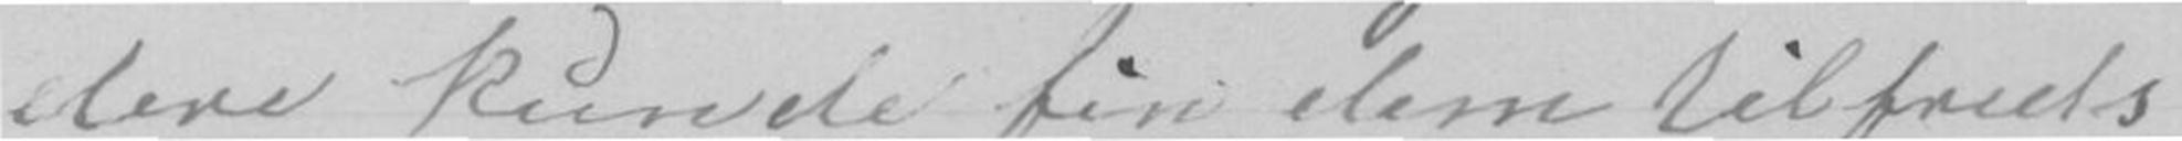dere kunde fin dem tilfreds
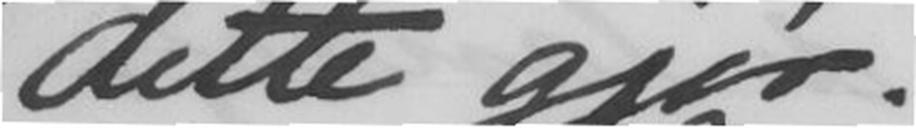dette gjør.
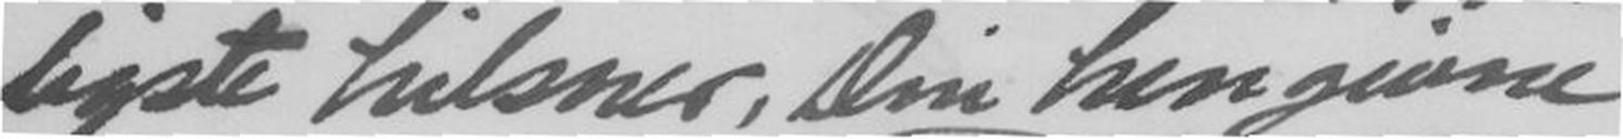ligste hilsner, Din hengivne
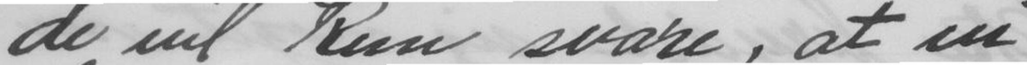de vil kun svare, at vi
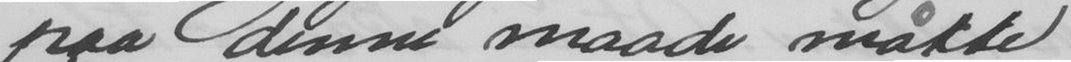paa denne maade måtte
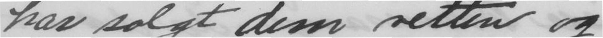har solgt dem retten og
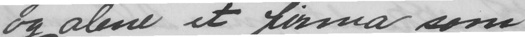og alene et firma som
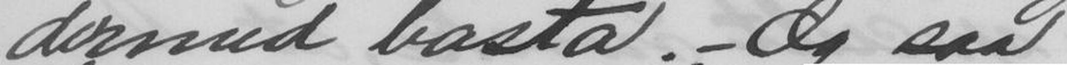dermed basta. - Og saa
